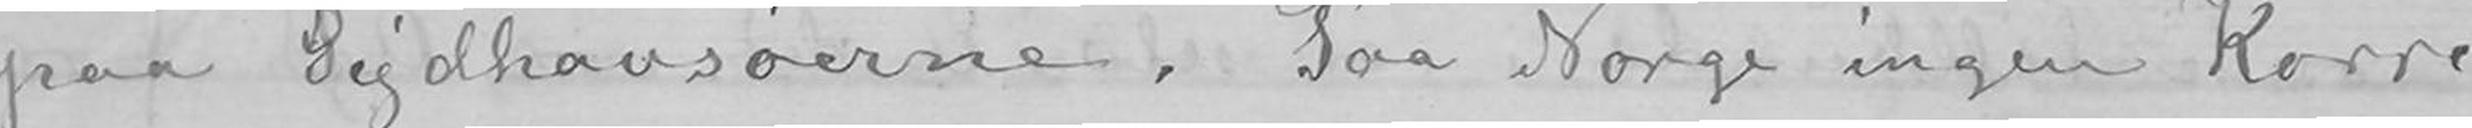paa Sydhavsøerne. Paa Norge ingen Korre-
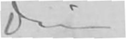Din
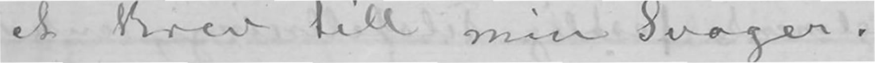et Brev till min Svoger.-
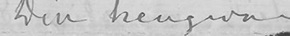Din hengivne
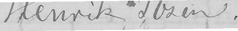Henrik Ibsen.
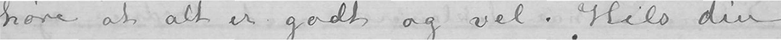høre at alt er godt og vel. Hils din
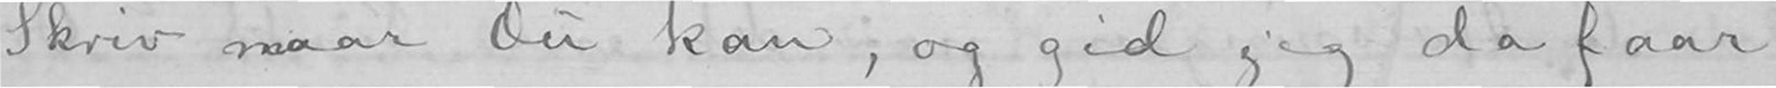Skriv naar Du kan, og gid jeg da faar
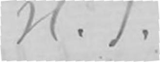H. I.
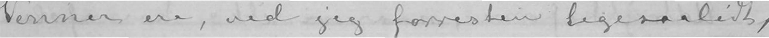Venner ere, ved jeg forresten ligesaalidt,
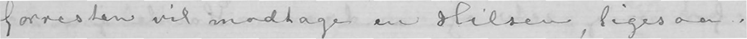forresten vil modtage en Hilsen, ligesaa.
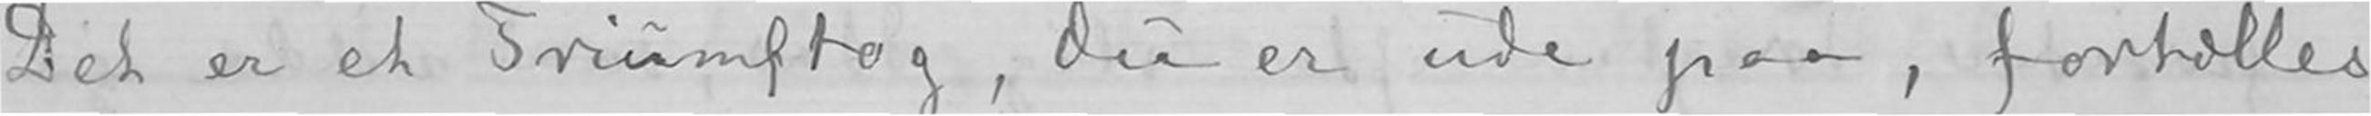Det er et Triumftog, Du er ude paa, fortælles
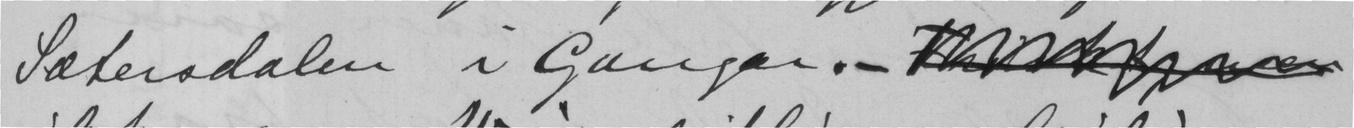Sætersdalen i Gangar. -
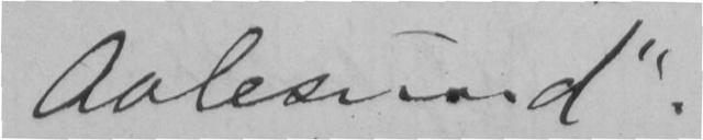Aalesund".
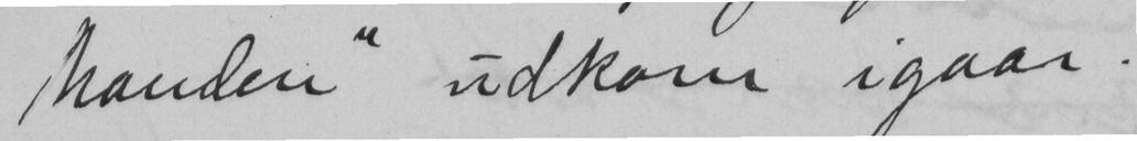Manden" udkom igaar.
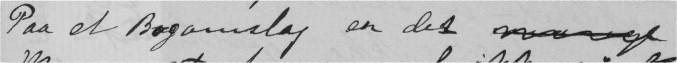Paa et Bogomslag er det
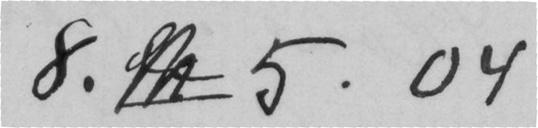8.  5.04
